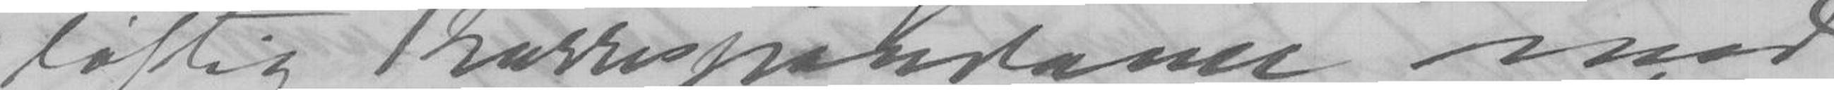løftig Korrespondance med
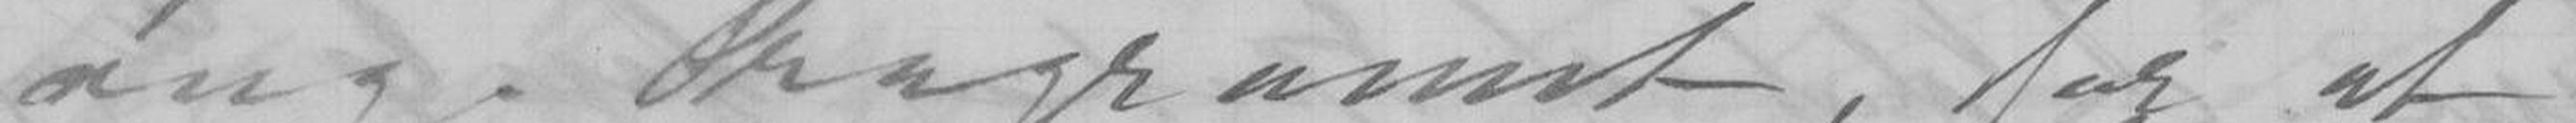ang. Programet, for at
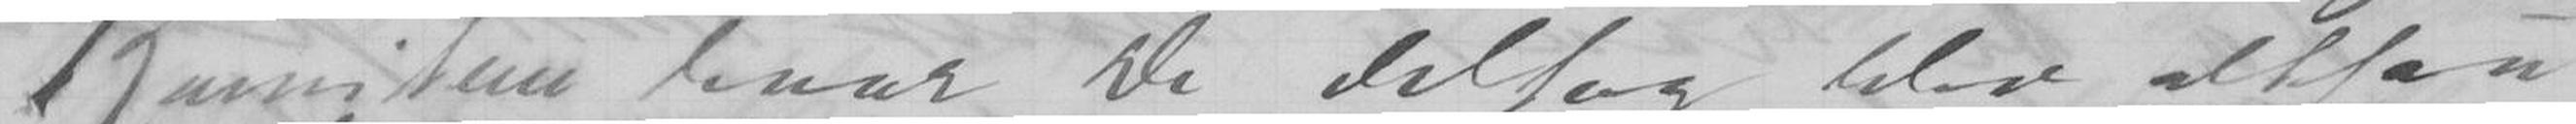Komiteen hvor De deltog blev altsaa
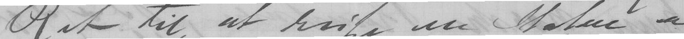Ret til at reise en Statue og
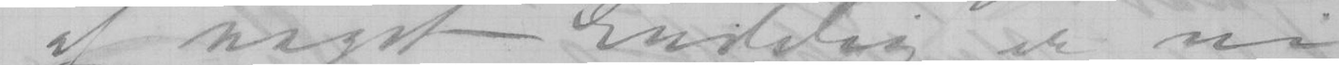af noget. Endelig er vi
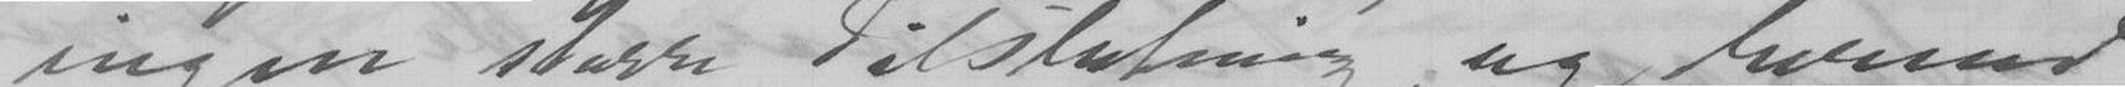ingen større Tilslutning, og herved
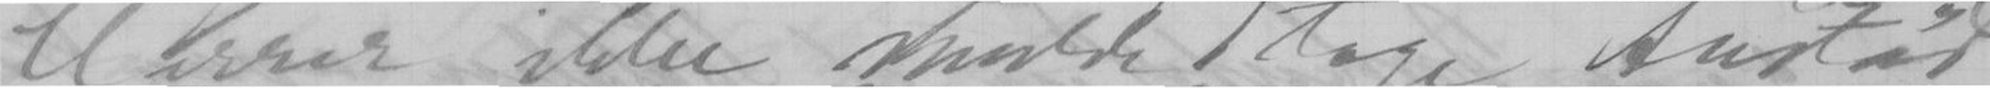Herrer ikke skulde tage Anstød
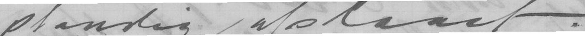stændig afslaaet.
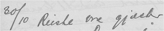30/10 Reiste vore gjæster
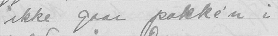ikke gaar pokke'n i
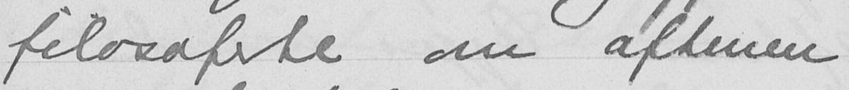filosoferte om aftenen
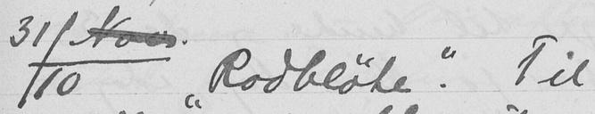31/10 "Rodbløte". Til
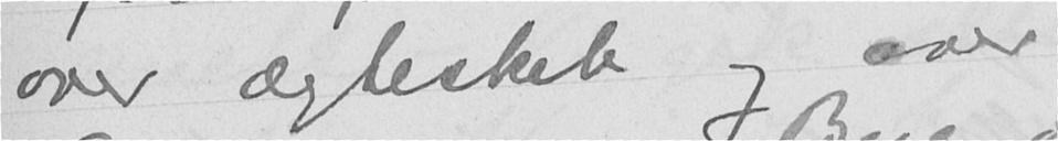over ægteskab og over
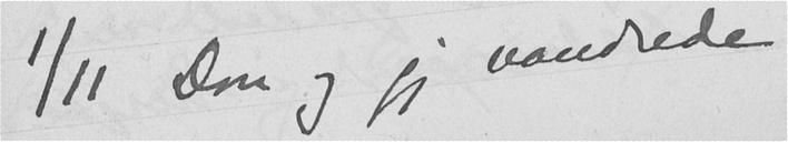1/11 Don og jeg vandrede
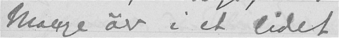Mange øer i et lidet
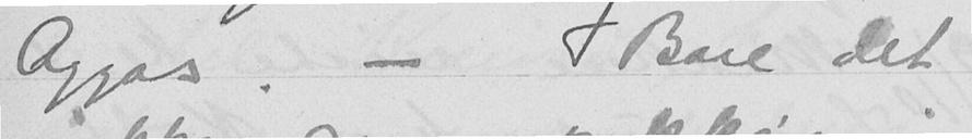Aggas. - Bare det
